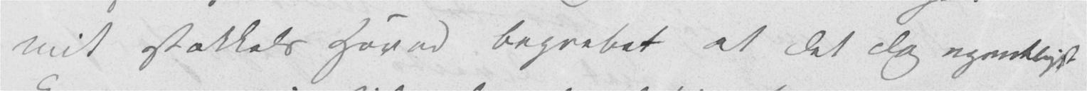mit Stakkels Hoved begrebet at det dog egentligst
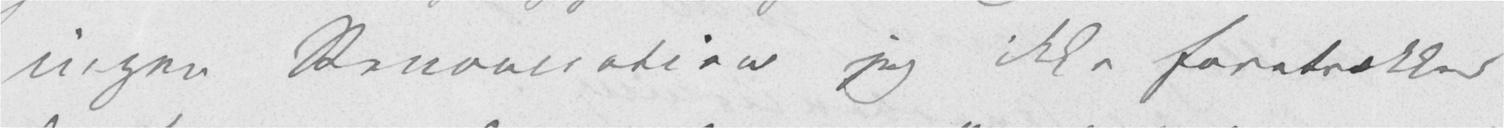ingen Renonciation jeg ikke foretrækker
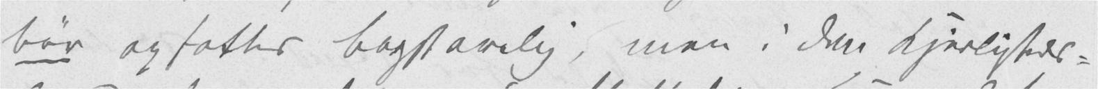bør opfattes bogstavelig, men i den Kjerligheds-
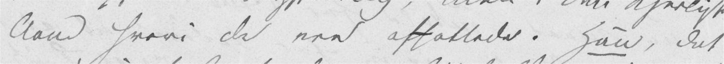Aand hvori de ere affattede. Han, det
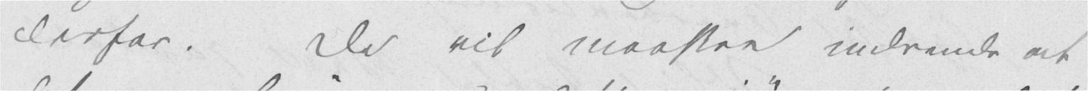derfor. De vil maaskee indvende at
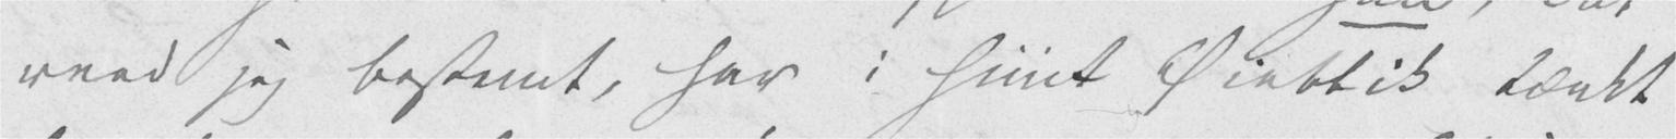veed jeg bestemt, har i hiint Øieblik tænkt
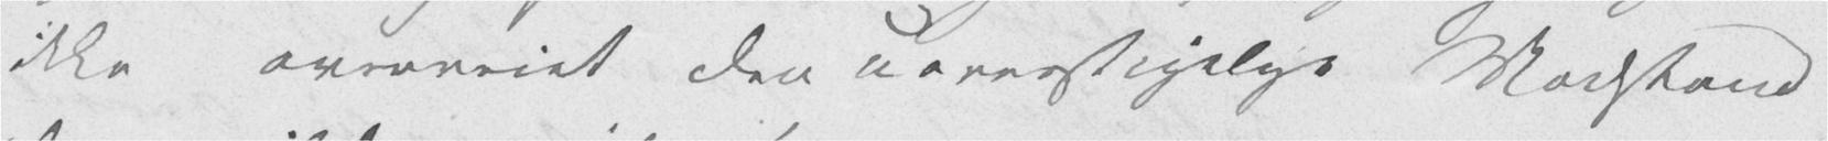ikke overveiet den uoverstigelige Modstand
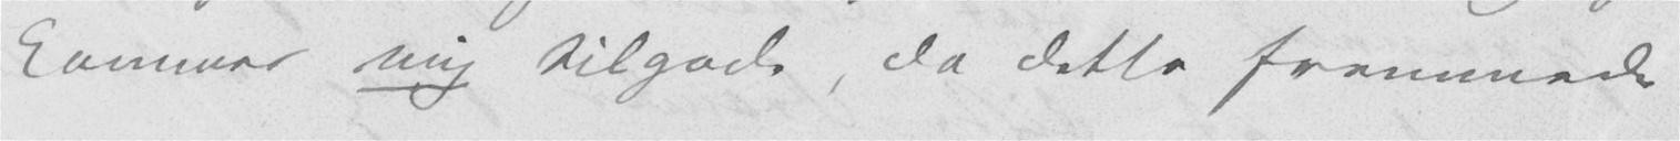kommer mig tilgode, da dette fremmede
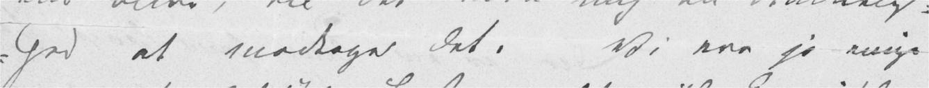hed at modtage det. Vi ere jo enige
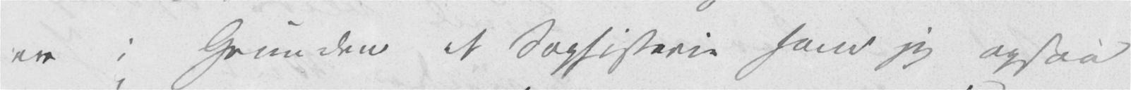er i Grunden et Sophisterie som jeg ogsaa
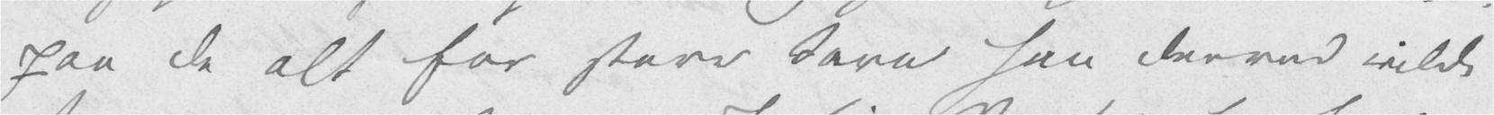paa de alt for store Savn han derved vilde
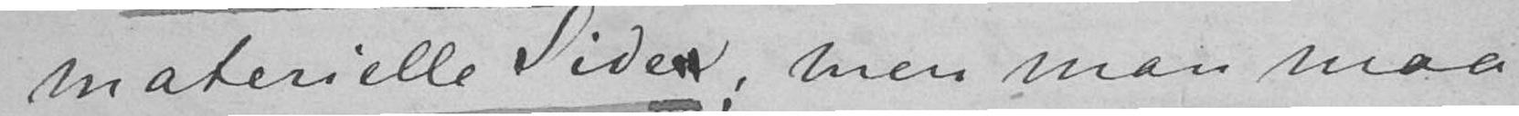materielle sider; men man maa
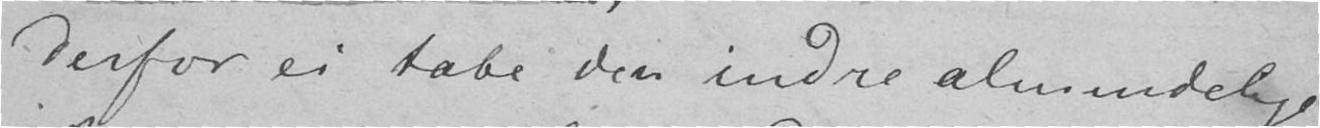derfor ei tabe den indre almindelige
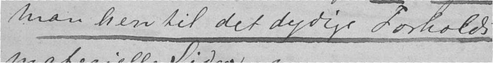man hen til det dydige Forholds
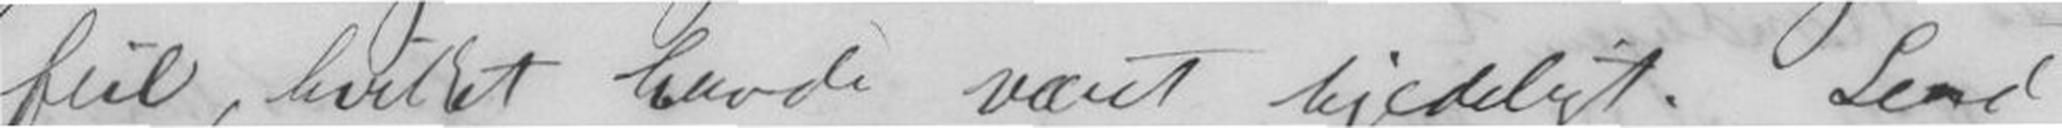feil, hvilket havde været kjedeligt. Send
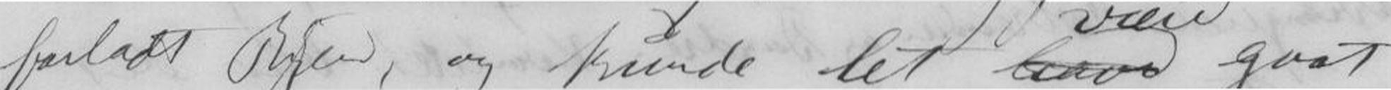forladt Byen, og kunde let have gaat
